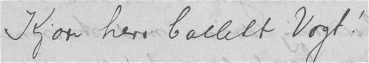Kjære herr Collett Vogt!
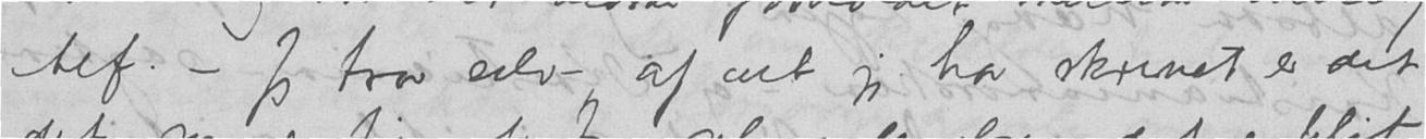Alf. - Jeg tror selv - af det jeg har skrevet er det
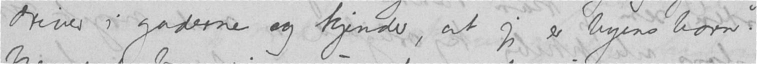driver i gaderne og kjender, at jeg er byens barn.
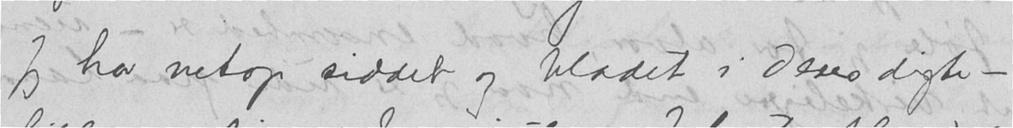Jeg har netop siddet og bladet i Deres digte -
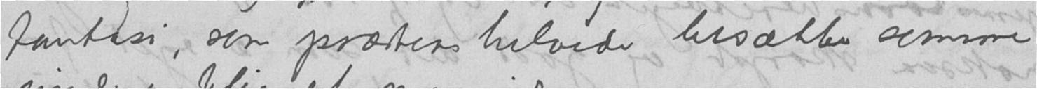fantasi, som præstens helvede besætter somme
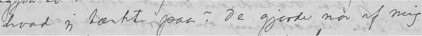hvad jeg tænkte paa? De gjorde nar af mig
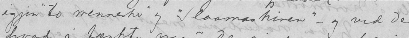igjen "to mennesker" og "Slaamaskinen" - og ved De
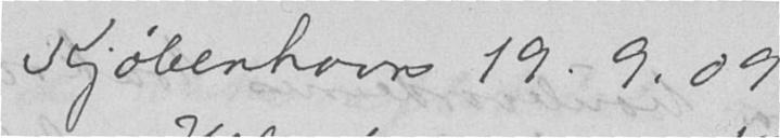Kjøbenhavn 19.9.09
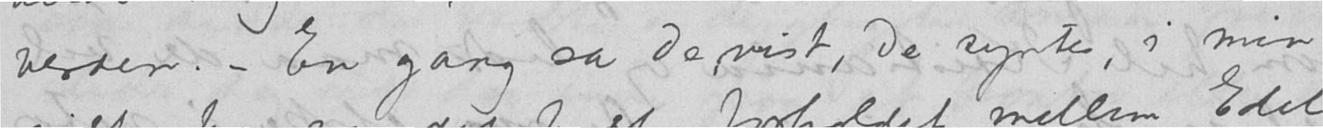verden. - En gang sa De vist, De syntes, i min
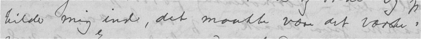bilder mig ind, det maatte være det værste i
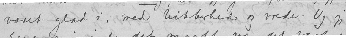været glad, i med bitterhed og vrede. Og jeg
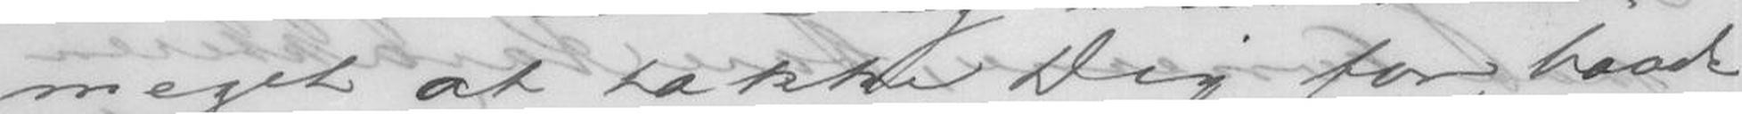meget at takke Dig for, baade
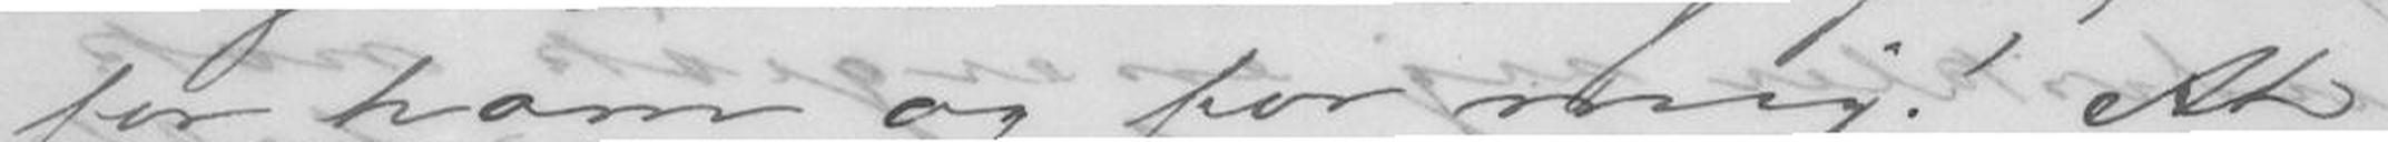for ham og for mig! At
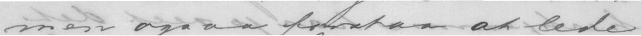men ogsaa forstaa at lede
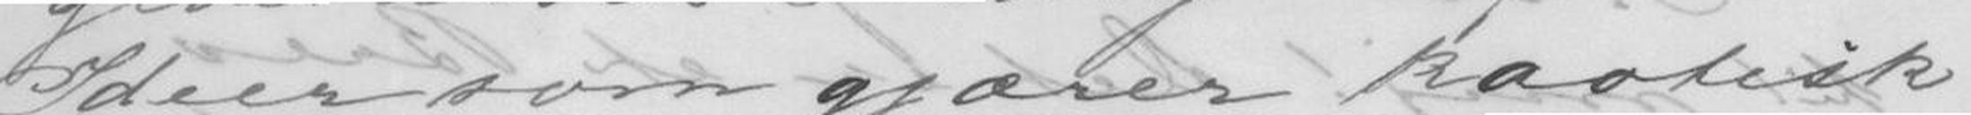Ideer som gjærer kaotisk
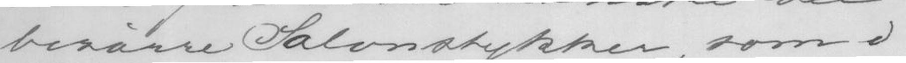bisarre Salonstykker, som i
